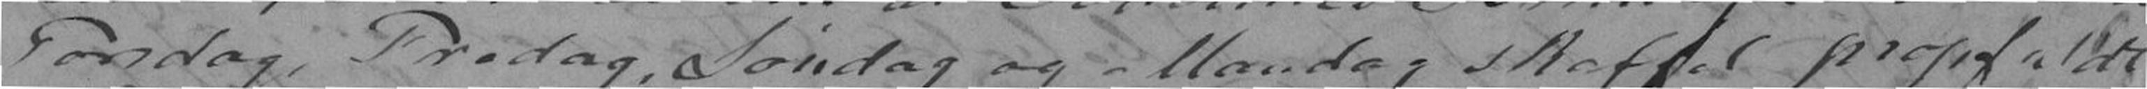Torsdag, Fredag, Søndag og Mandag skaffet propfuldt
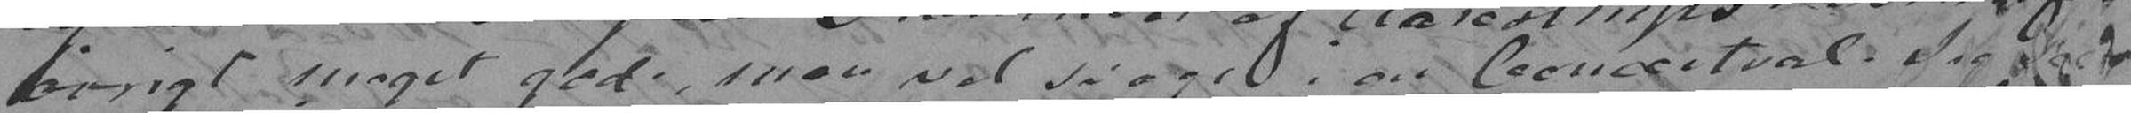øvrigt meget gode, man vel svage i en Concertsal. Jeg saa
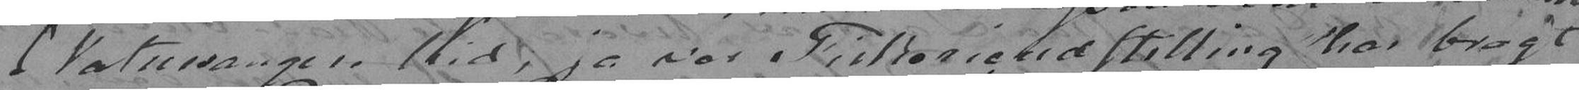Natursanger hid, ja vor Fiskerieudstilling har bragt
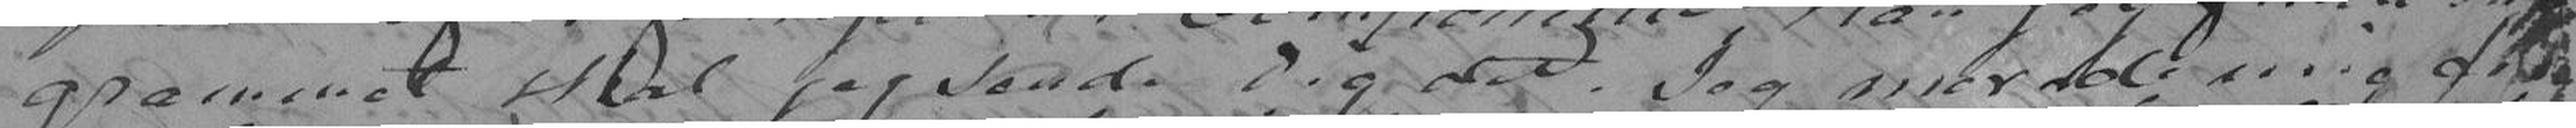grammet skal jeg sende Dig det. Jeg morede mig for-
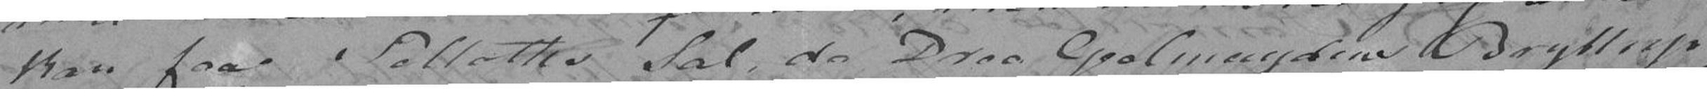kan faae Polloths Sal, da Drea Geelmuydens Bryllup
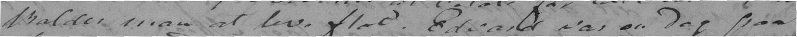kalder man at leve flot. Edvard var en Dag paa
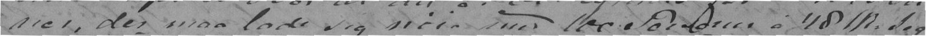ner, der maa lade sig nøie med 100 Personer à 48 Sk. Jeg
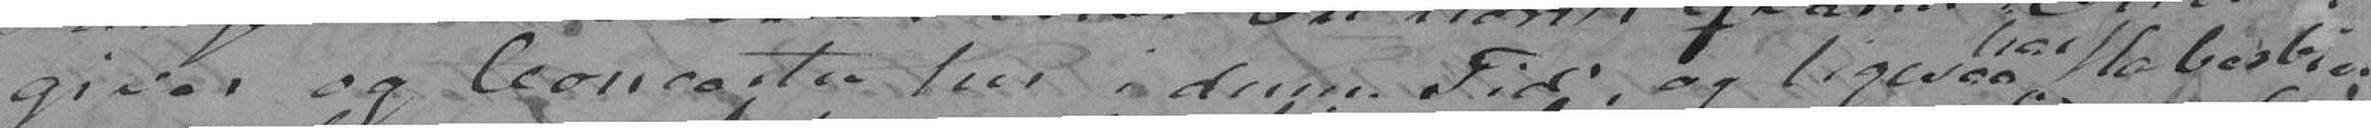giver og Concerter her i denne Tid, og ligesaa har Haberbier
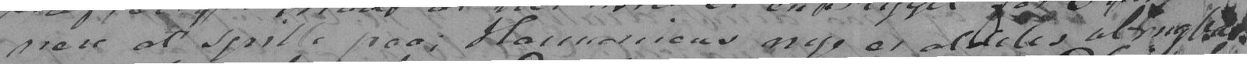nere at spille paa; Harmoniens nye er aldeles ubrugbar
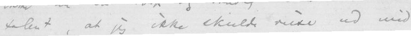talent, at jeg ikke skulde reise ud méd
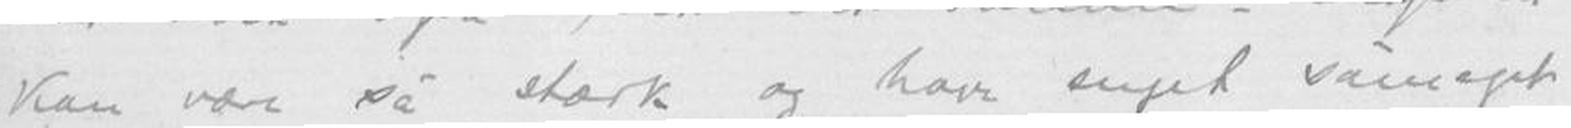kan være så stærk og have suget såmeget
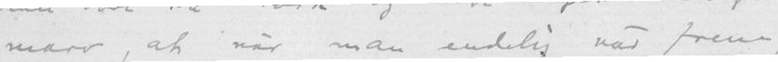marv, at når man endelig når frem,
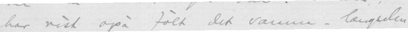har vist også følt det samme - længselen
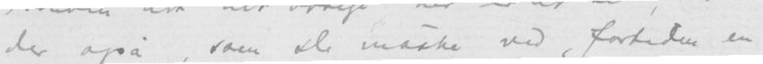der også, som De måske ved, fortiden en
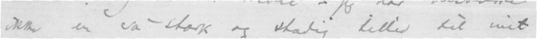ikke en så stærk og stadig tillid til mit
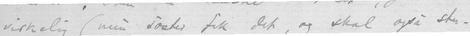virkelig (min søster fik det, og skal også stu-
